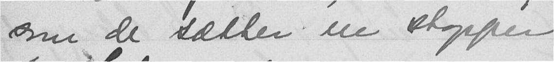som de sætter en stopper
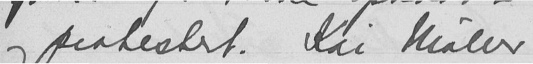og protestert. Kai Møller
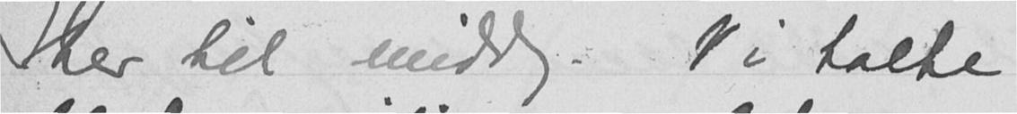her til middag. Vi talte
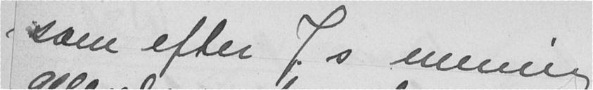som efter J.s mening
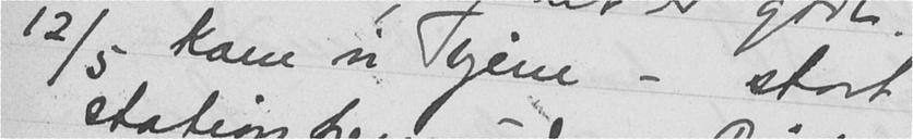12/5 Kom vi hjem - stort
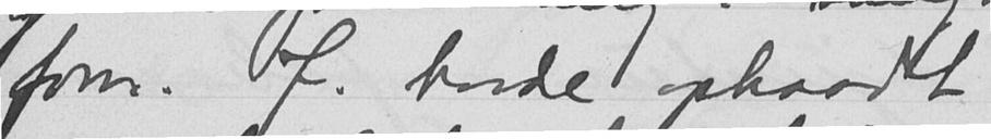forn. J. havde opholdt
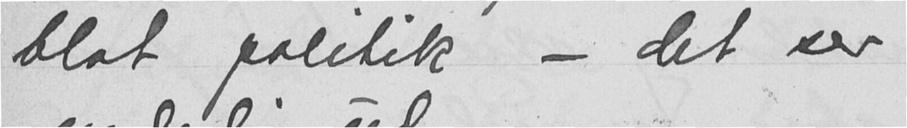blot politik - det ser
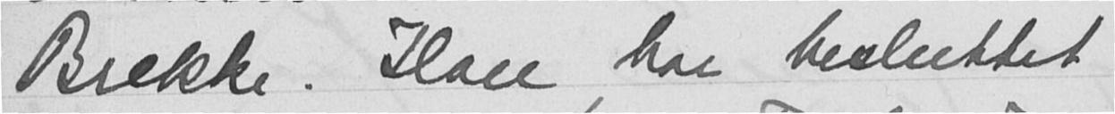Brekke. Han har besluttet
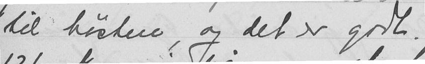til høsten, og det er godt.
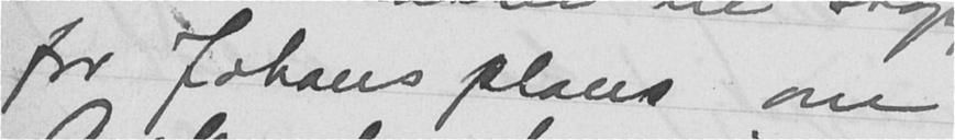for Johans plan om
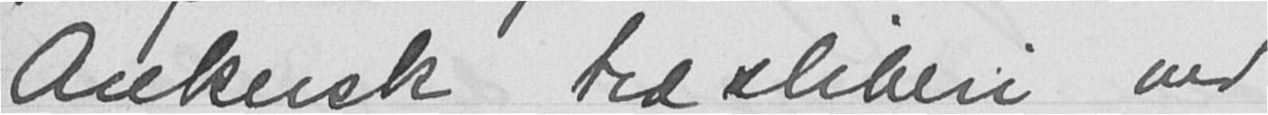Ankersk træsliberi ved
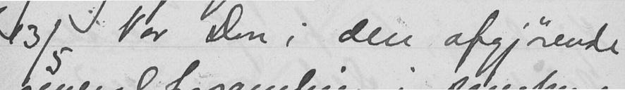13/5 Var Don i den afgjørende
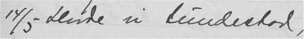14/5 Havde vi Lundestad,
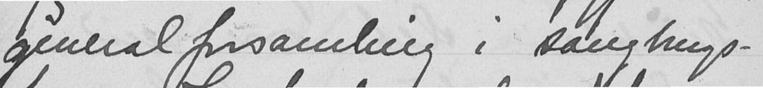generalforsamling i saugbrugs-
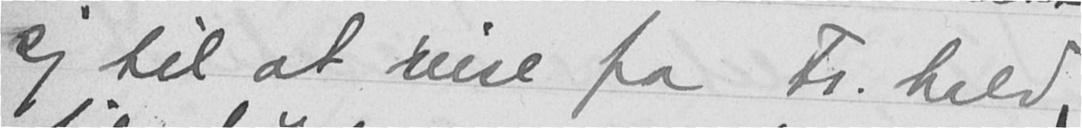sig til at reise fra Fr. hald
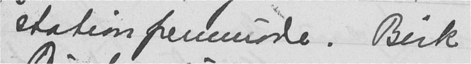stationfremmøde. Birk
